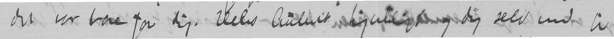det var bare for dig. Hils Aubert hjerteligt og dig selv med li
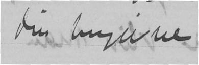din hengivne
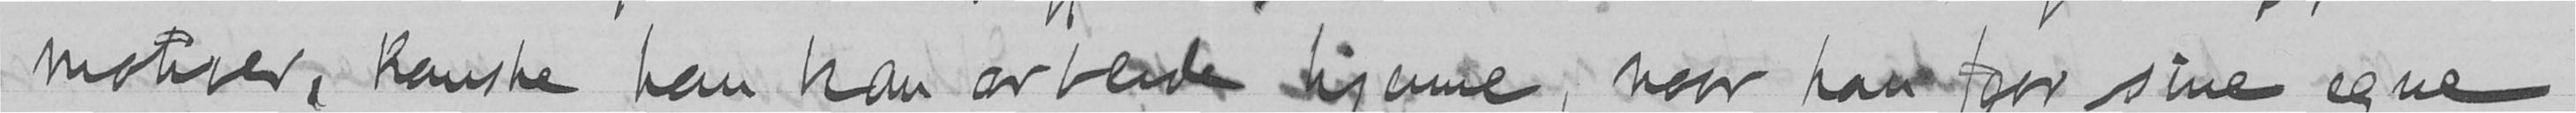motiver, kanske han kan arbeide hjemme, naar han faar sine egne
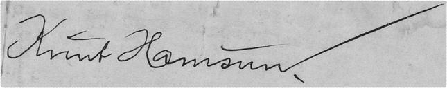Knut Hamsun.
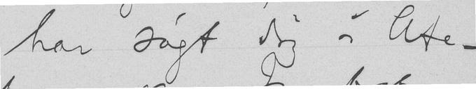har søgt dig i Ate-
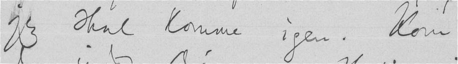jeg skal komme igen. Kom
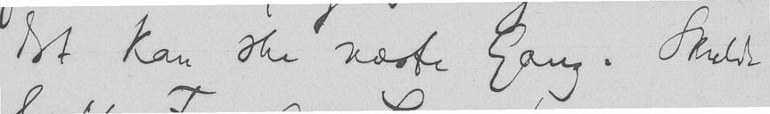det kan ske næste Gang. Skulde
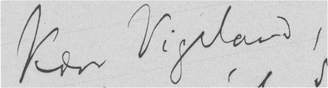Kære Vigeland,
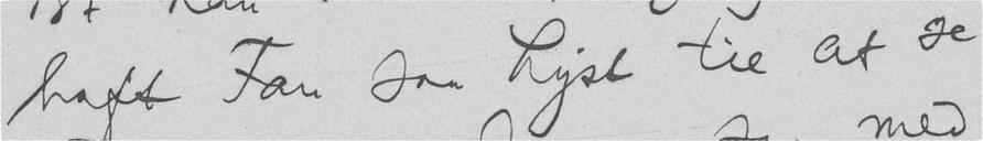haft Fan saa Lyst til at se
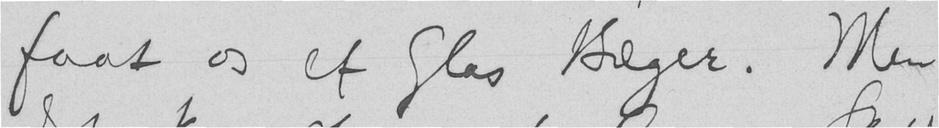faat os et Glas Bæger. Men
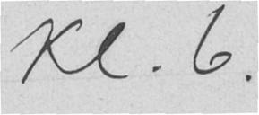Kl. 6.
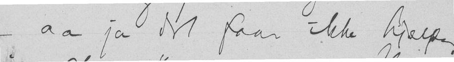- aa ja det faar ikke hjelpe,
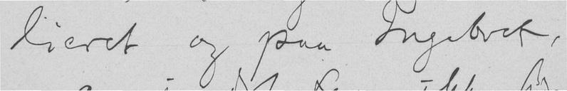lieret og paa Ingebret,
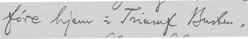føre hjem i Triumf Busten.
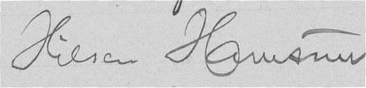Hilsen Hamsun
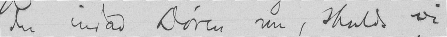du indad Døren nu, skulde vi
